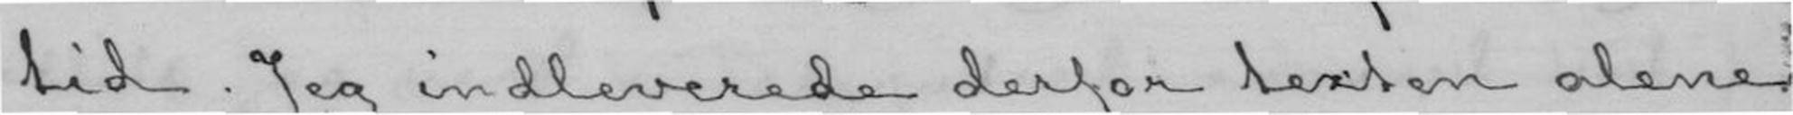tid. Jeg indleverede derfor texten alene
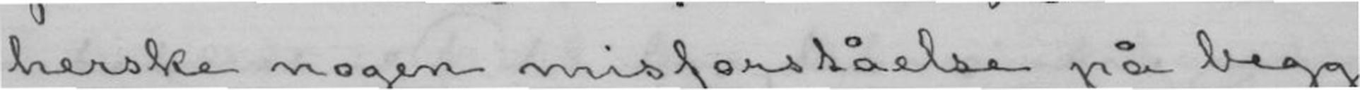herske nogen misforståelse på begge
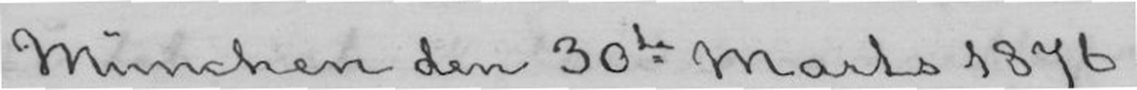München den 30te Marts 1876.
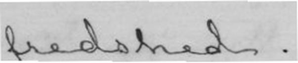fredshed.
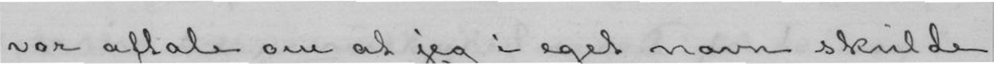vor aftale om at jeg i eget navn skulde
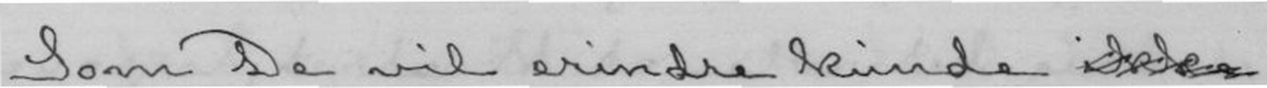Som De vil erindre kunde ikke
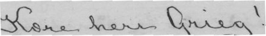Kære herr Grieg!
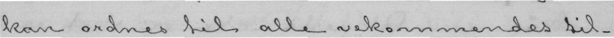kan ordnes til alle vedkommendes til-
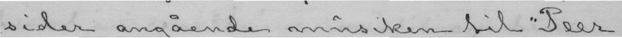sider angående musiken til "Peer
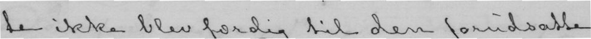te ikke blev færdig til den forudsatte
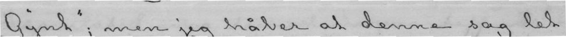Gynt"; men jeg håber at denne sag let
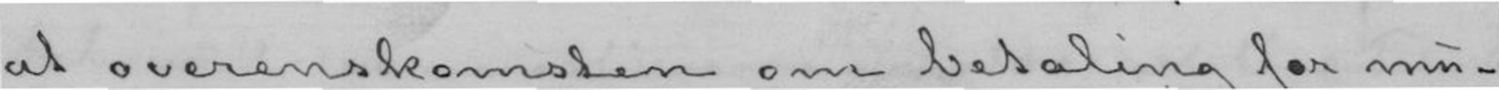at overenskomsten om betaling for mu-
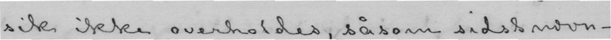sik ikke overholdes, så som sidst nævn-
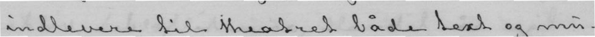indlevere til theatret både text og mu-
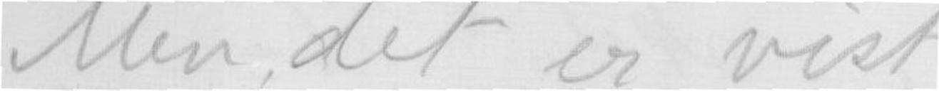Men det er vist
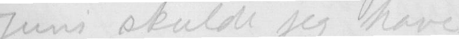Juni skulde jeg have
